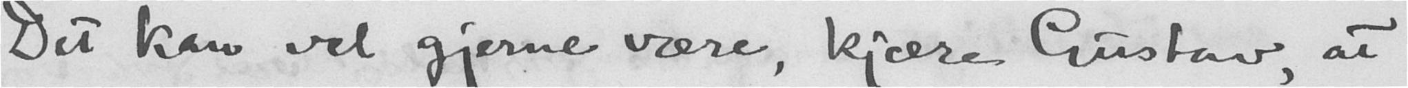Det kan vel gjerne være, kjære Gustav, at
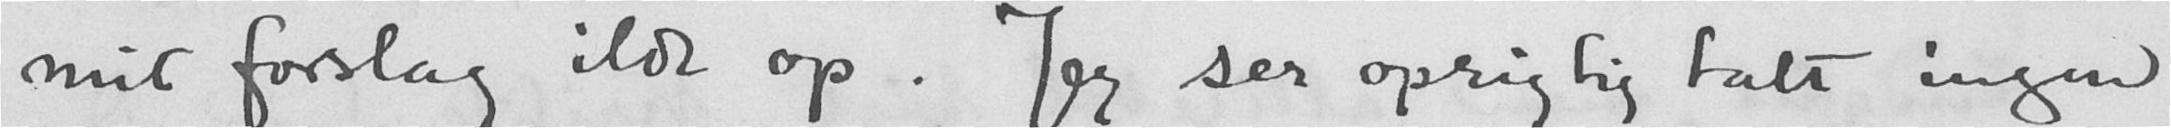mit forslag ilde op. Jeg ser oprigtig talt ingen
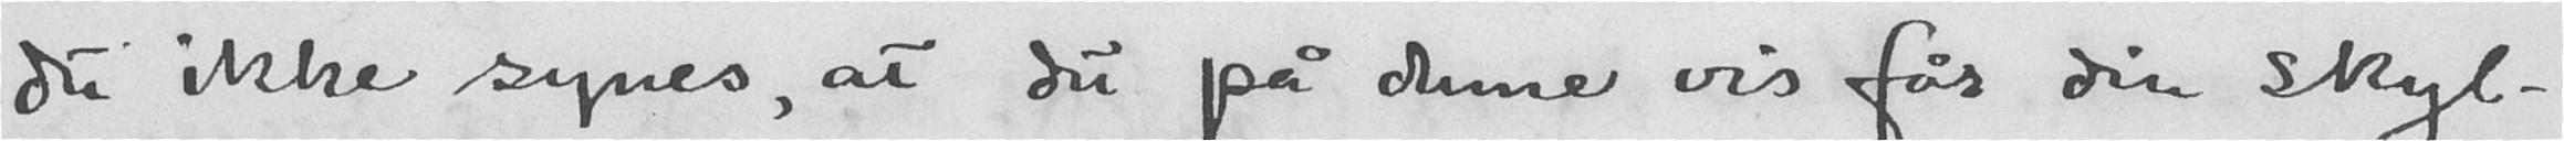du ikke synes, at du på denne vis får din skyl-
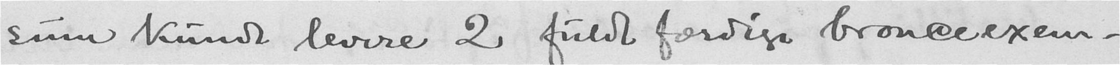sum kunde levere 2 fuldt færdige bronceexem-
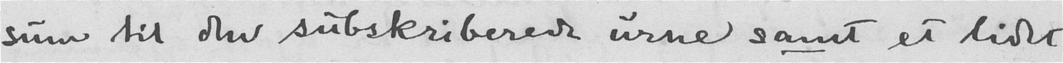sum til den subskriberede urne samt et lidet
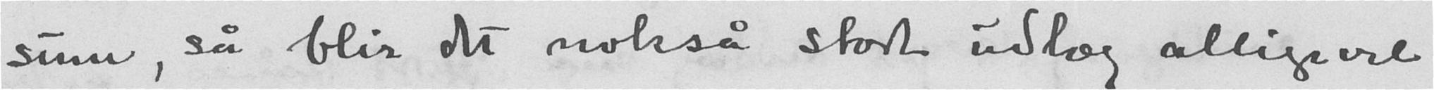sum, så blir det nokså stort udlæg alligevel
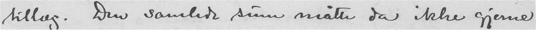tillæg. Den samlede sum måtte da ikke gjerne
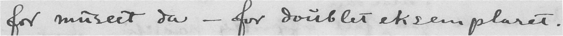for museet da - for doublet eksemplaret.
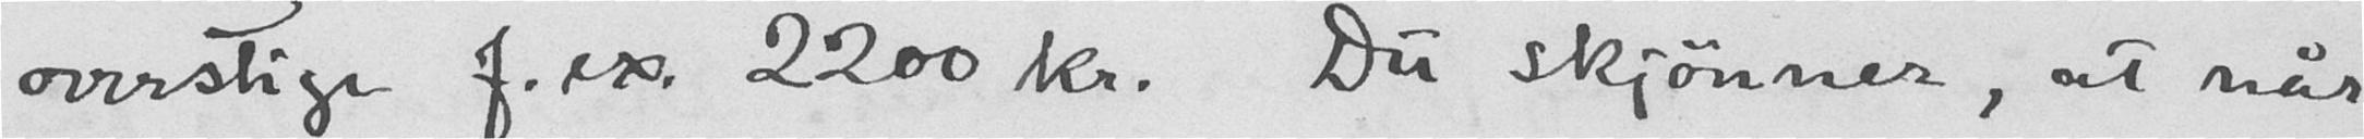overstige f. ex 2200 Kr. Du skjønner, at når
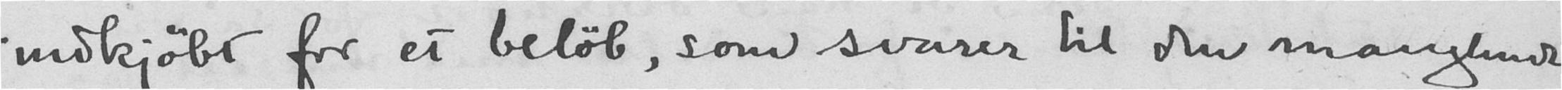indkjøbt for et beløb, som svarer til den manglende
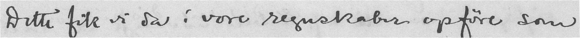Dette fik vi da i vore regneskaber opføre som
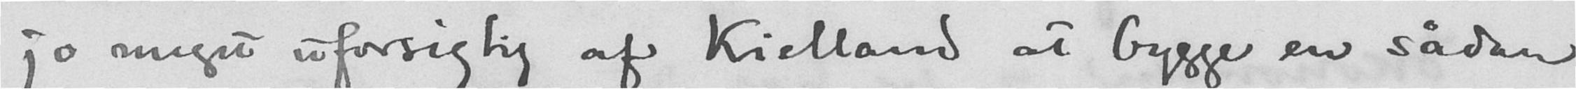jo meget uforsigtig af Kielland at bygge en sådan
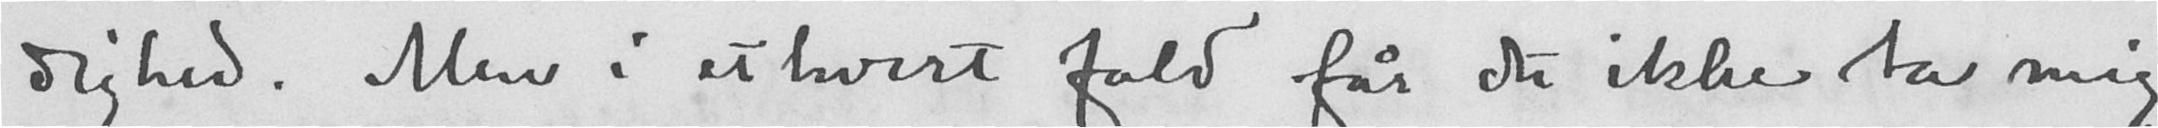dighed. Men i ethvert fald får du ikke ta mig
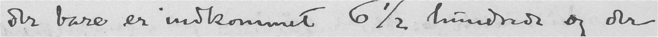der bare er indkommet 6 ½  hundrede og der
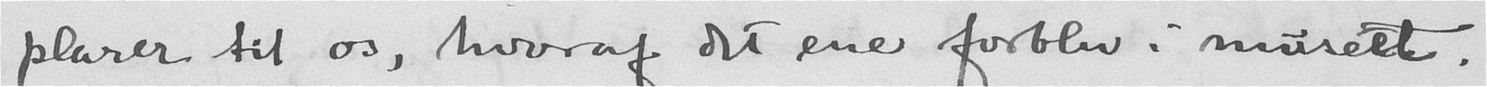plarer til os, hvoraf det ene forblev i museet.
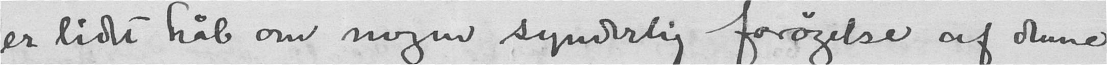er lidet håb om nogen synderlig forøgelse af denne
